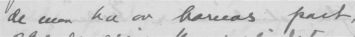de maa ha av barnas part,
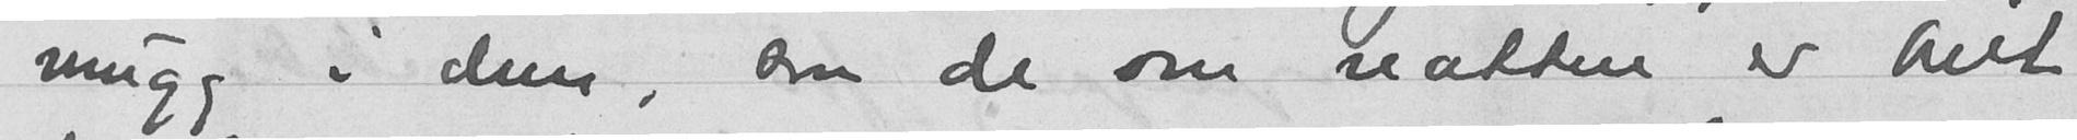mugg i dem, saa de om natten er blit
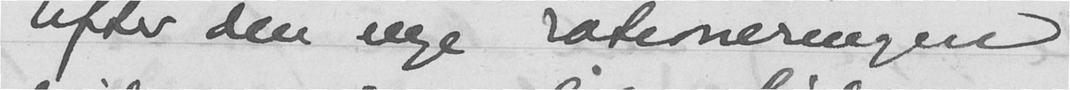Efter den nye rationeringen
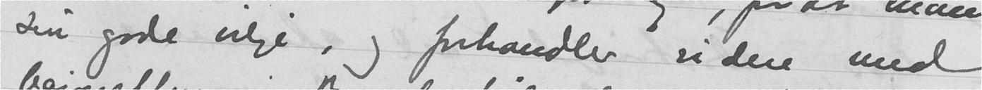sin gode vilje, og forhandler siden med
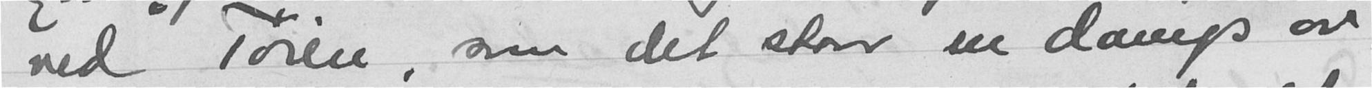ved Tøien, som det staar en damps av,
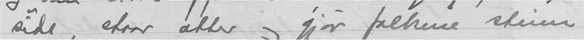side, staar atter og gjør folkene stum
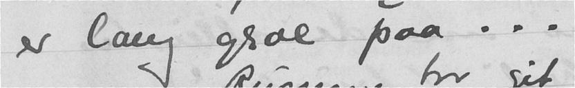er lang gral paa...
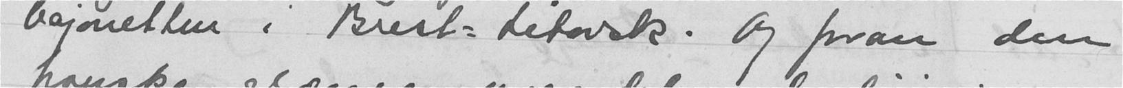bajonetten i Brest-Litovsk. Og foran den
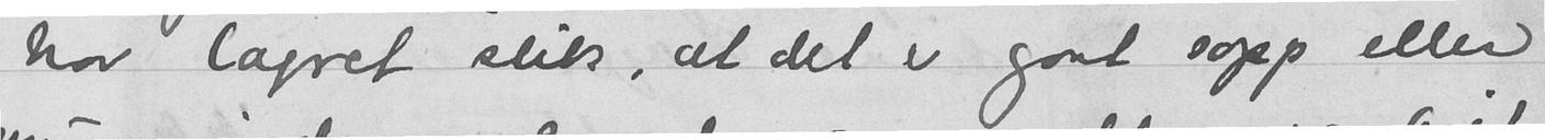har lagret slik, at det er gaat sopp eller
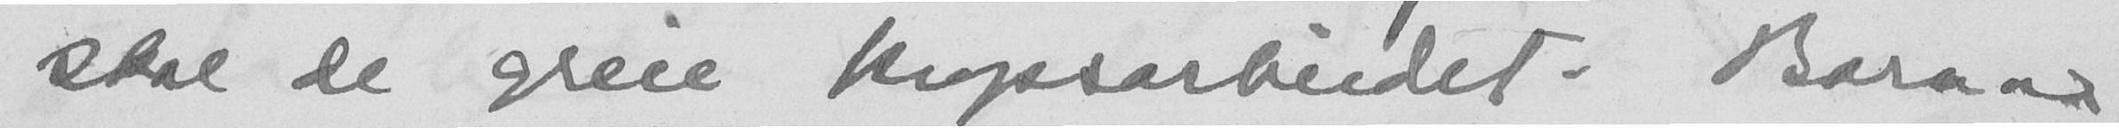skal de greie kropsarbeidet. Barna
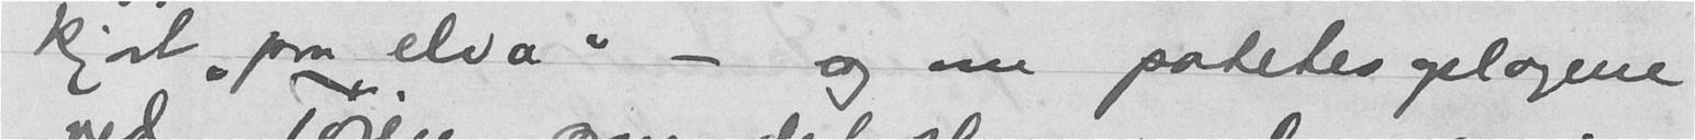kjørt "paa elva" - og om potetes oplagene
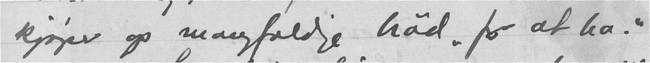kjøper op mangfoldige brød "for at ha."
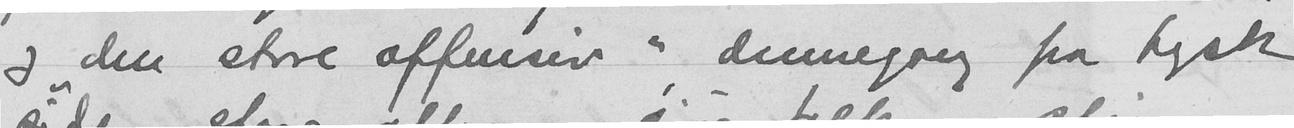og "den store offensiv", dennegang fra tysk
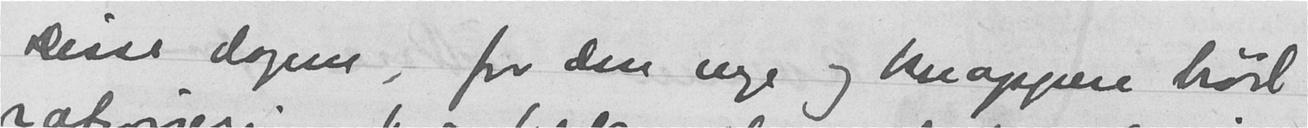Disse dagene, for den uge og knappere brød
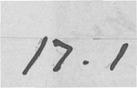17.1
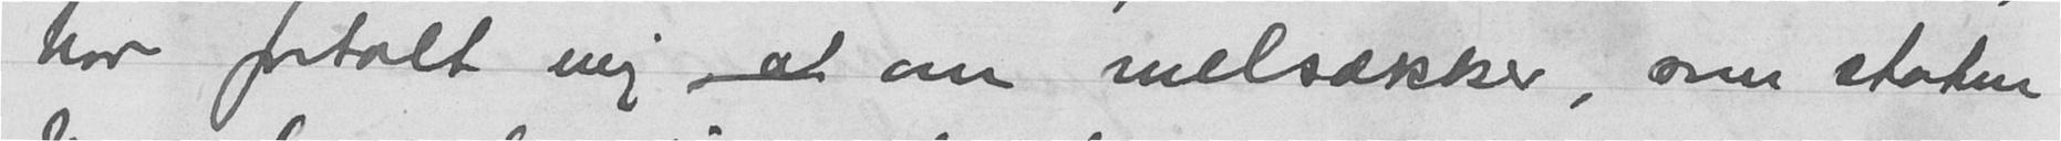har fortalt mig at om melsækker, som staten
